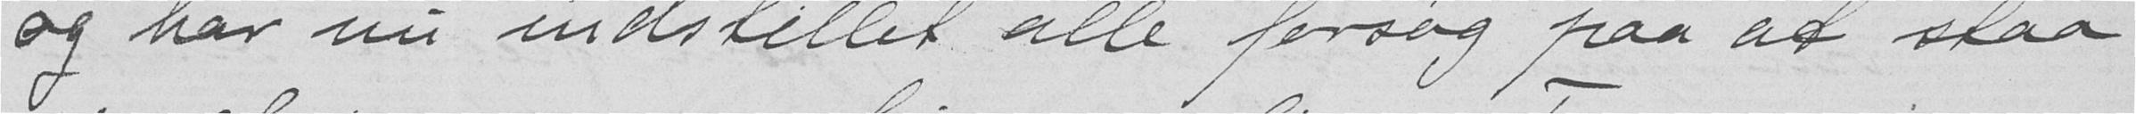og har nu indstillet alle forsøg paa at staa
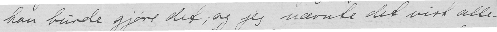han burde gjøre det; og jeg nævnte det vist alle-
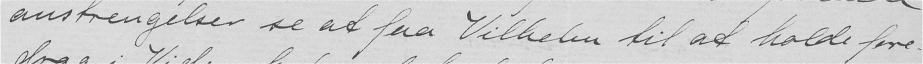anstrengelser se at faa Vilhelm til at holde fore-
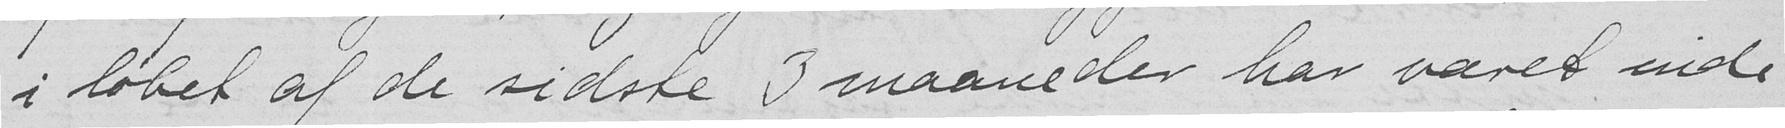i løbet af de sidste 3 maaneder har været inde
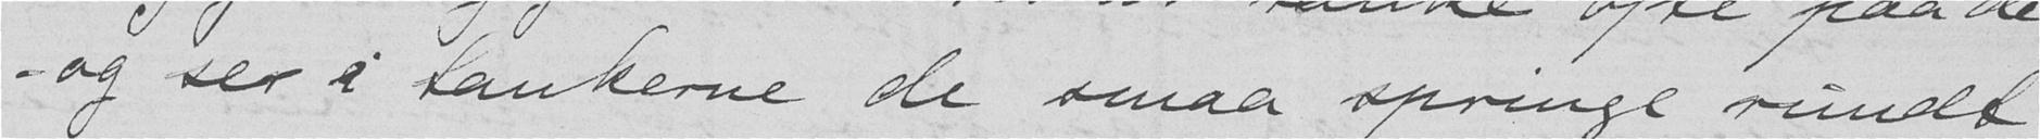– og ser i tankerne de smaa springe rundt
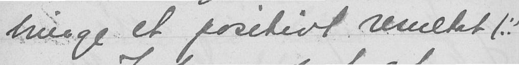bringe et positivt resultat!!
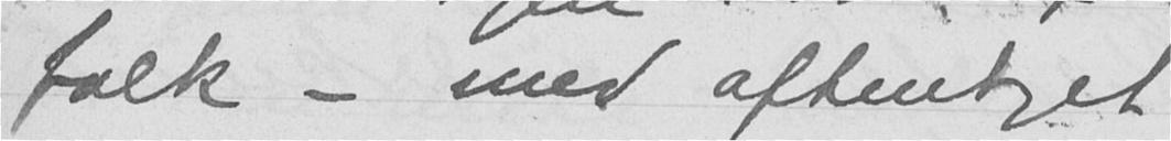folk - med aftentoget
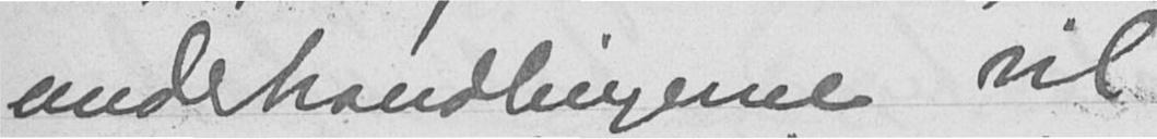underhandlingerne vil
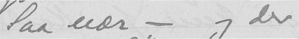Saa nær - og der
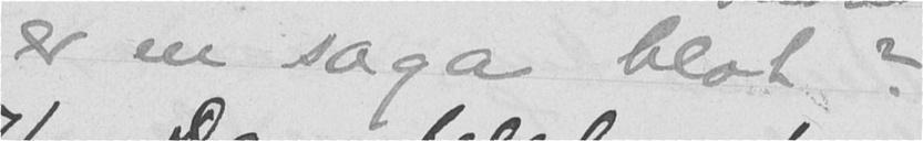er en saga blot?
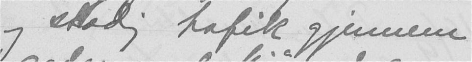og stadig trafik gjennem
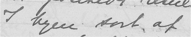I byen sort af
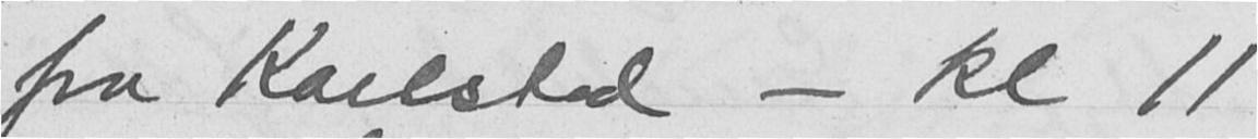fra Karlstad - kl 11
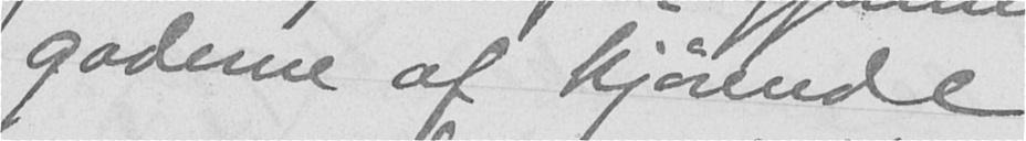gaderne af kjørende
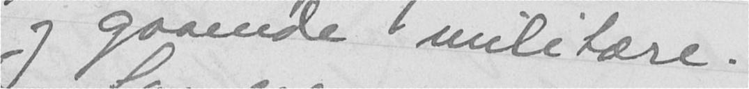og gaaende militære.
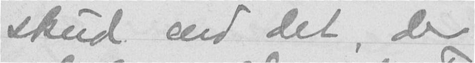skud end det, der
